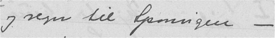og regn til Sponvigen -
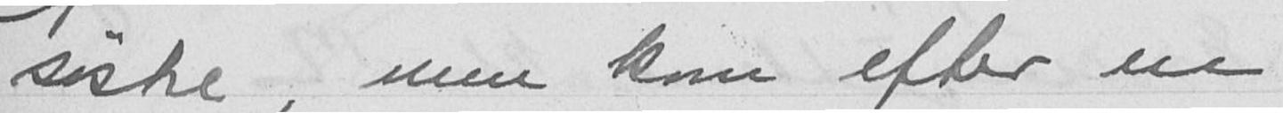søstre, men kom efter en
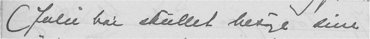(Julie har skullet besøge sine
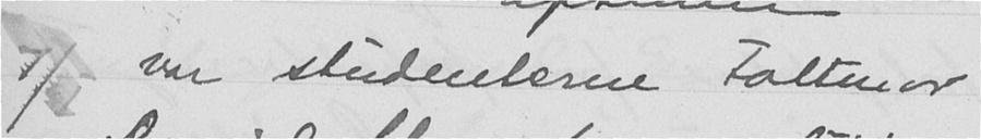7/I var studenterne Foltmar
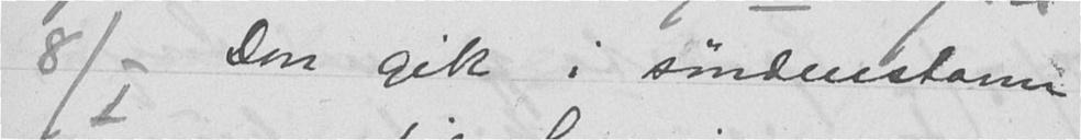8/I Don gik i søndenstorm
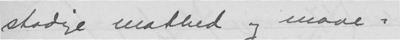stadige mathed og mave-
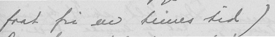faat fri en times tid)
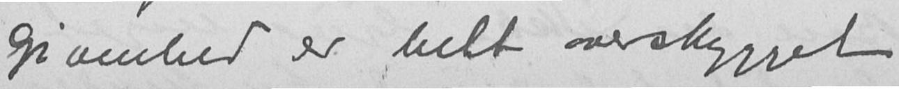givenhed er helt overskygget
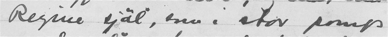Regine sjøl, som i stor pomps
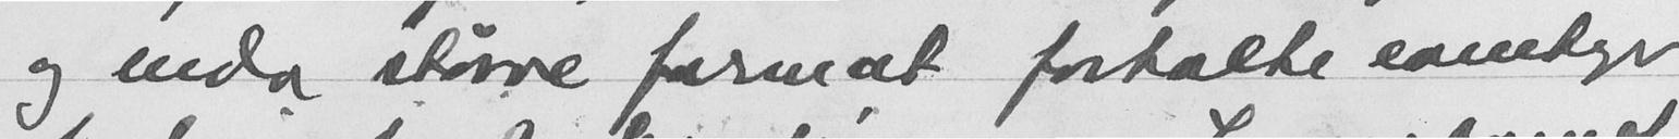og enda større format fortalte eventyr
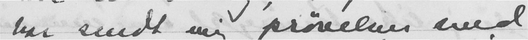har sendt mig prøvelsen med
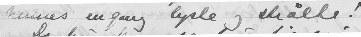hennes en gang lyste og strålte!
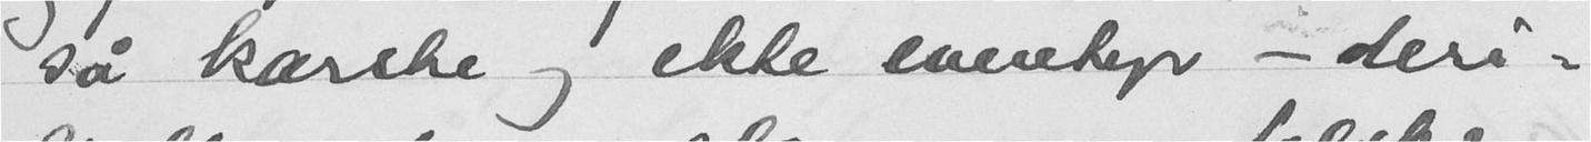så karske og ekte eventyr - deri-
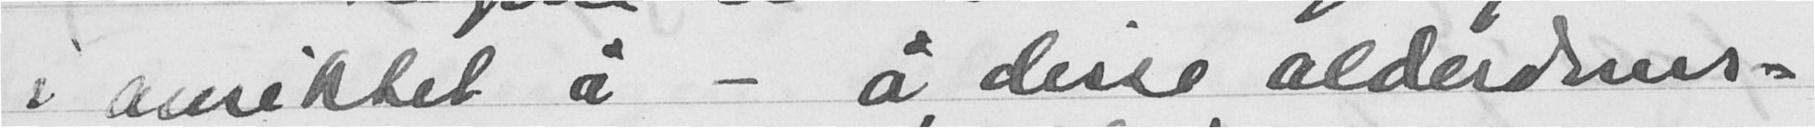i ansiktet å - å disse alderdoms-
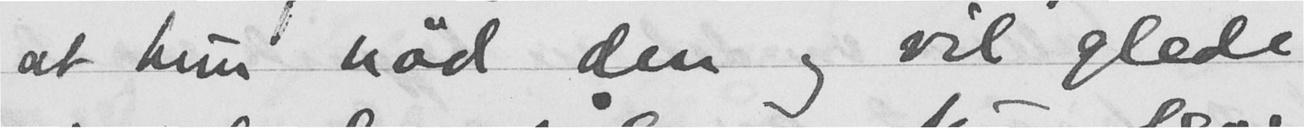at hun nød den og vil glede
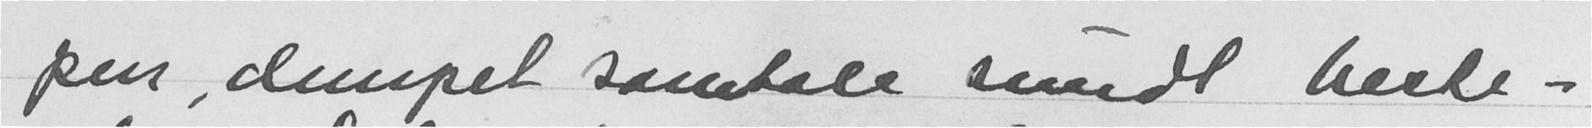pen, dempet samtale rundt heste-
